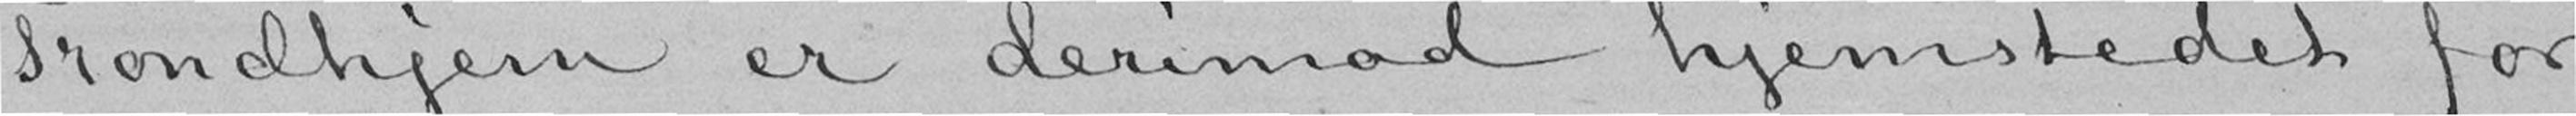Trondhjem er derimod hjemstedet for
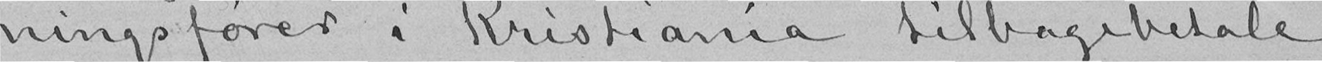ningsfører i Kristiania tilbagebetale
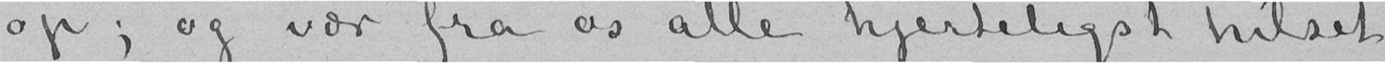op; og vær fra os alle hjerteligst hilset
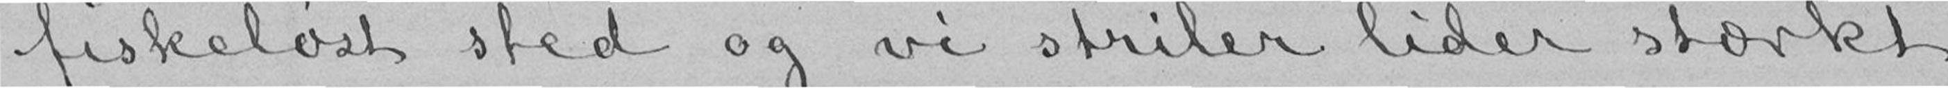fiskeløst sted og vi striler lider stærkt
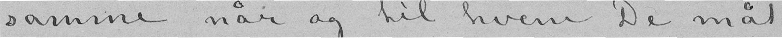samme når og til hvem De måt-
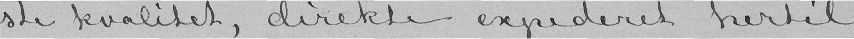ste kvalitet, direkte expederet hertil
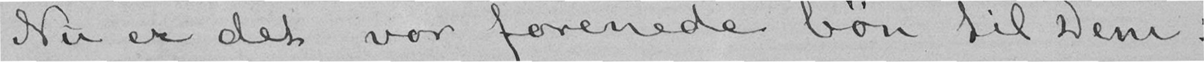Nu er det vor forenede bøn til Dem:
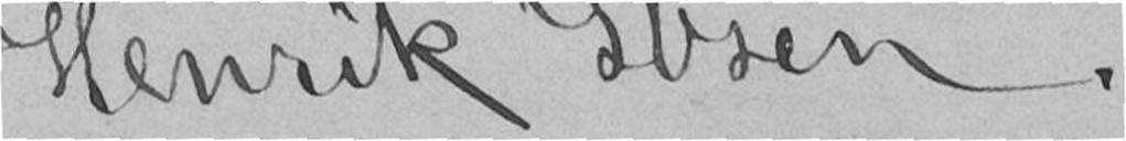Henrik Ibsen.
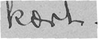kært.
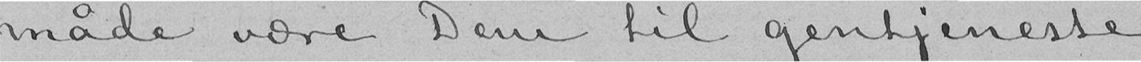måde være Dem til gentjeneste
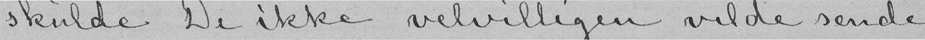skulde De ikke velvilligen vilde sende
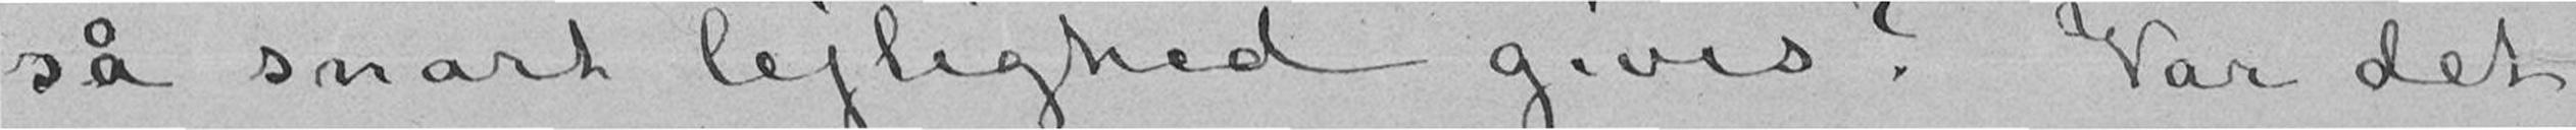så snart lejlighed gives? Var det
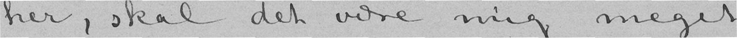her, skal det være mig meget
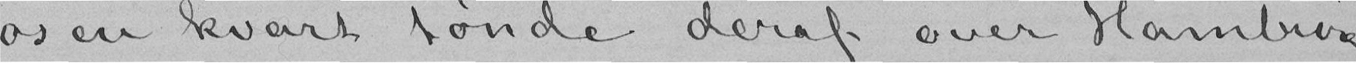os en kvart tønde deraf over Hamburg
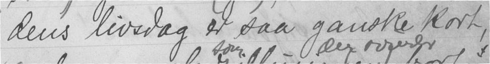dens livsdag er saa ganske kort,
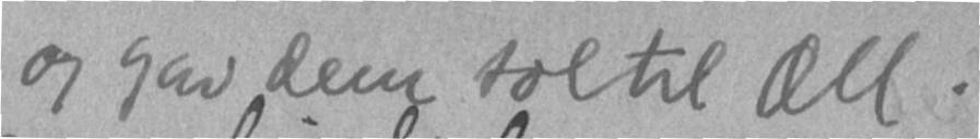og gav dens sol til æll.
